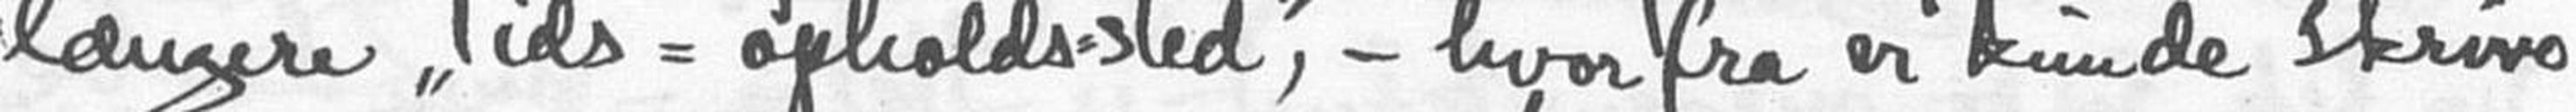længere "tids-opholds-sted", - hvorfra vi kunde skrive
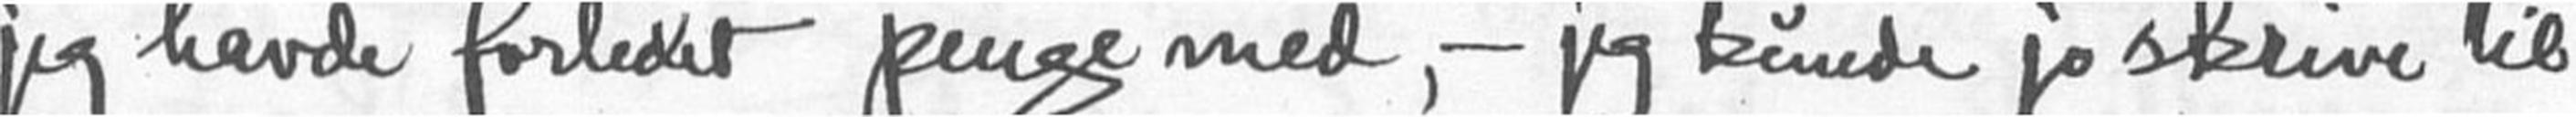jeg havde forlidet penge med, - jeg kunde jo skrive til
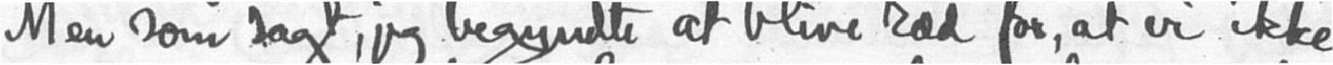Men som sagt, jeg begyndte at blive ræd for, at vi ikke
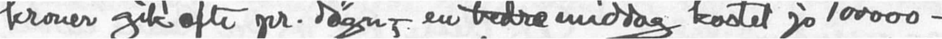kroner gik ofte pr. døgn, - en bedre middag kostet jo 100 000 -
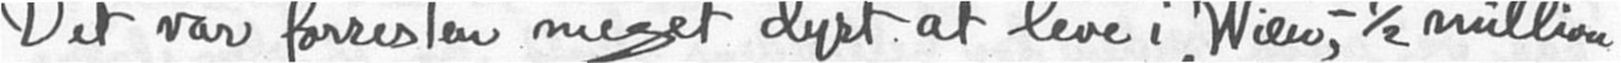Der var forresten meget dyrt at leve i Wien, - 1/2 million
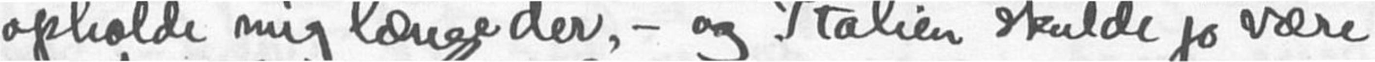opholde mig længe der, - og Italien skulde jo være
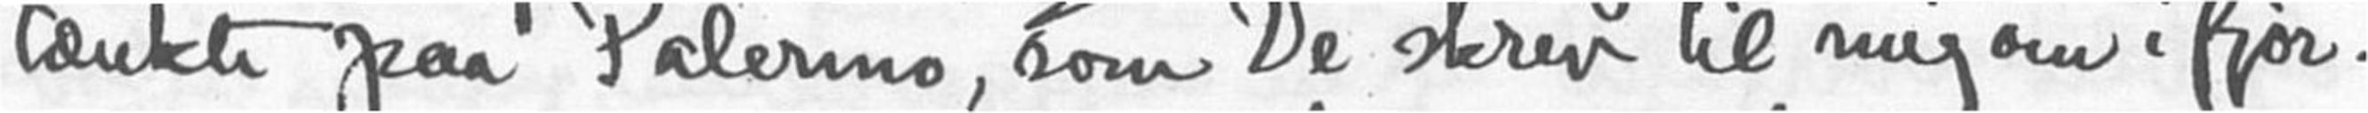tænkte paa Palermo, som De skrev til mig om ifjor.
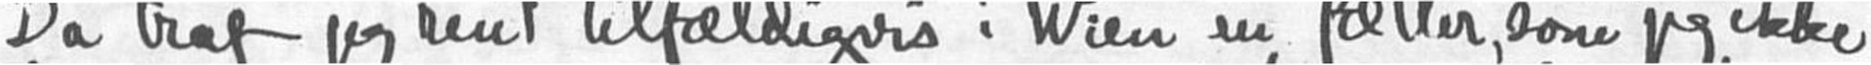Da traf jeg rent tilfeldigvis i Wien en fetter, som jeg ikke
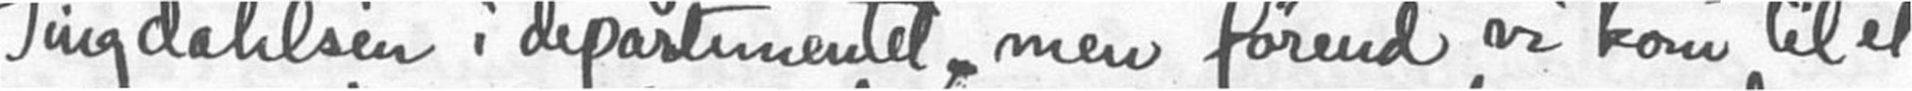Singdahlsen i departementet, men førend vi kom til et
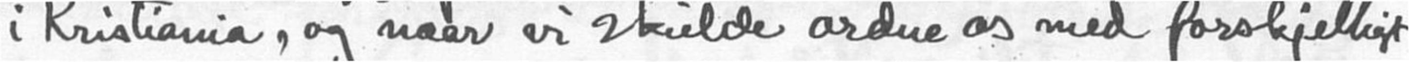i Kristiania, og naar vi skulde ordne os med forskjelligt
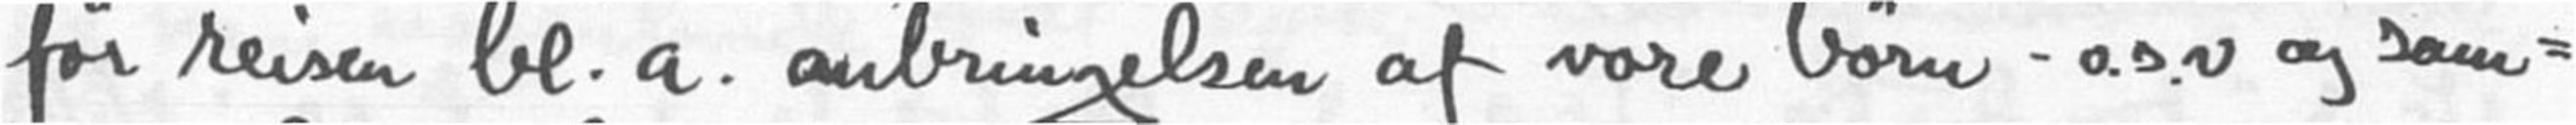for reisen bl. a. anbringelsen af vore børn o.s.v og sam-
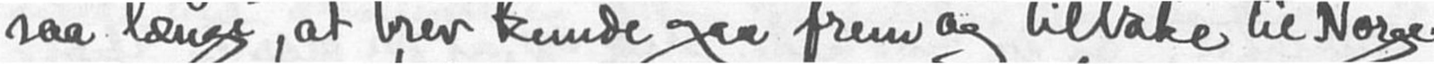saa længe, at brev kunde gaa frem og tilbage til Norge.
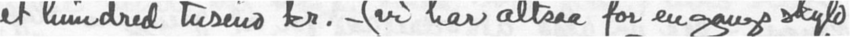et hundred tusen kr. - (vi har altså for en gangs skyld
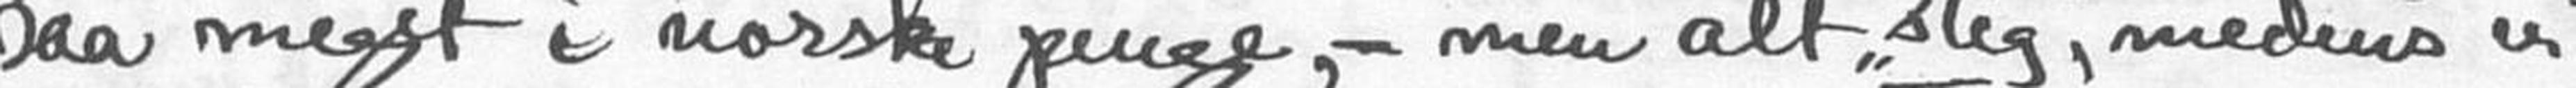saa meget i norske penge, - men alt "steg" medens vi
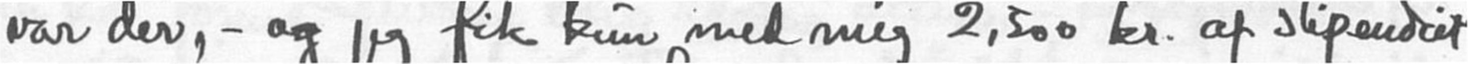var der, - og jeg fik kun med mig 2500 kr. af stipendet
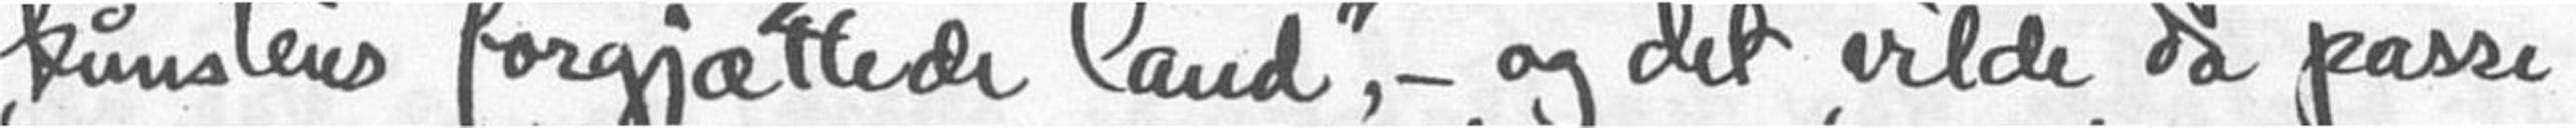"kunstens forgjættede land", - og det vilde da passe
